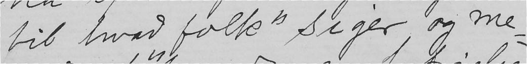til hvad "folk" siger og me-
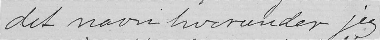det navn hvorunder jeg
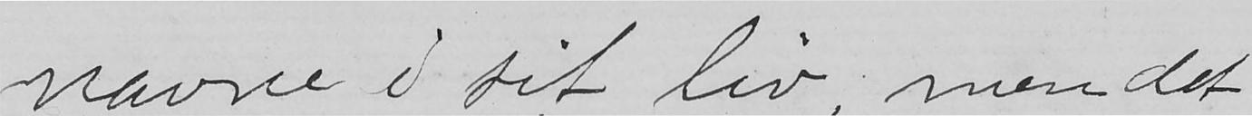navne i sit liv, men det
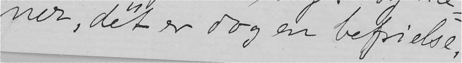ner, det er dog en befrielse.
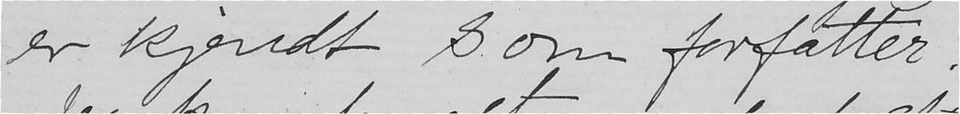er kjendt som forfatter.
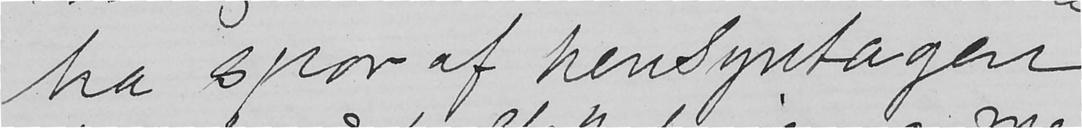ha spor af hensyntagen
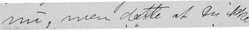nu, men dette at Du ikke
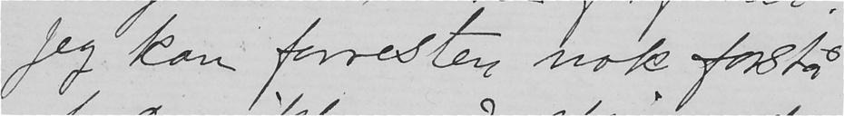Jeg kan forresten nok forstå
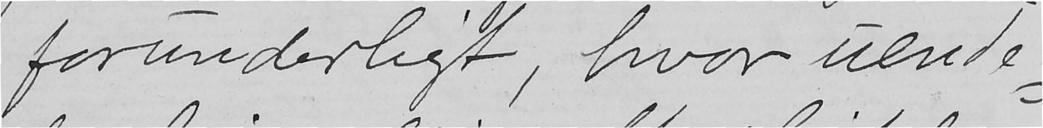forunderligt, hvor uende-
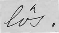løs.
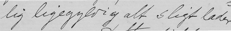lig ligegyldig alt sligt lader
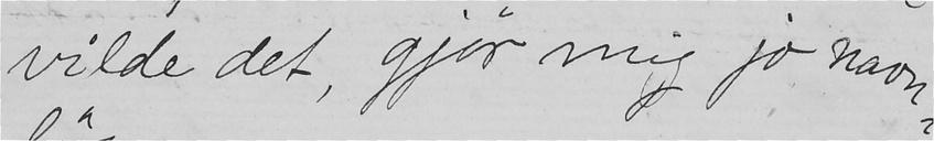vilde det, gjør mig jo navn-
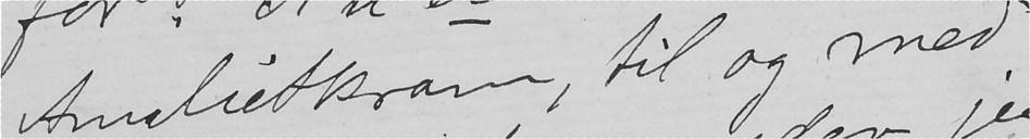Amalie Skram, til og med
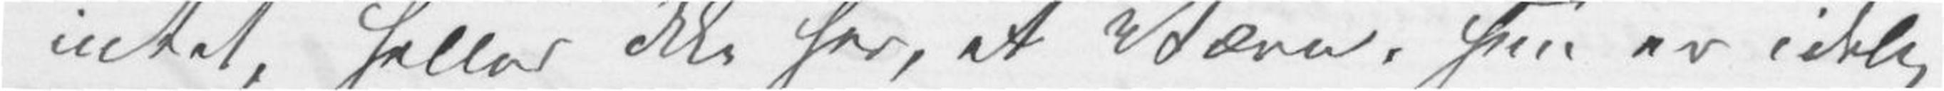intet, heller ikke her, et Værn, hun er idelig
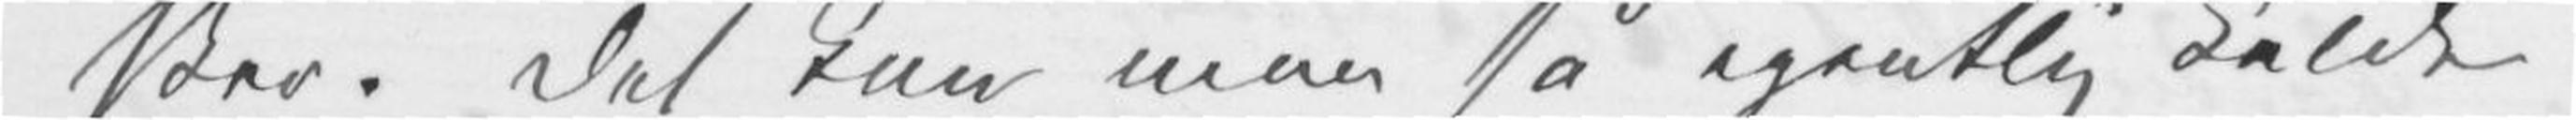sker. Det kan man så egentlig kalde
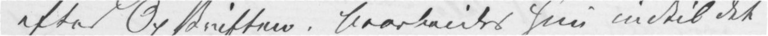efter Opskriften, bearbeides hun indtil det
